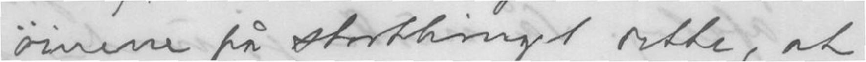øinene på stortinget dette, at
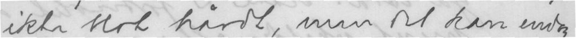ikke blot hårdt, men det kan endog
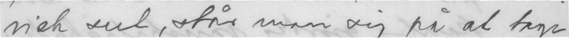risk seet, står man sig på at tage
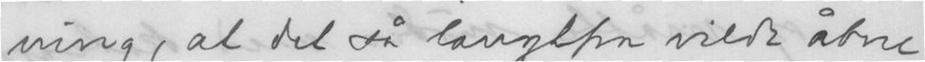ning, at det så langtfra vilde åbne
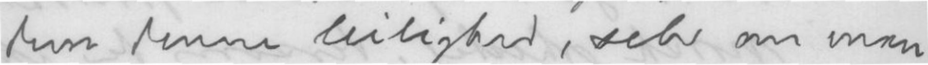Dem denne leilighed, selv om man
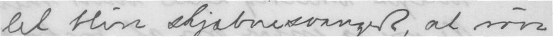let blive skjæbnesvangert, at røve
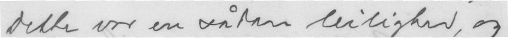Dette var en sådan leilighed, og
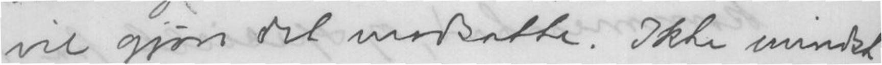vil gjøre det modsatte. Ikke mindst
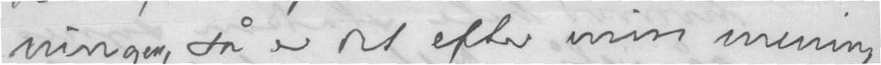ningen, så er det efter min mening
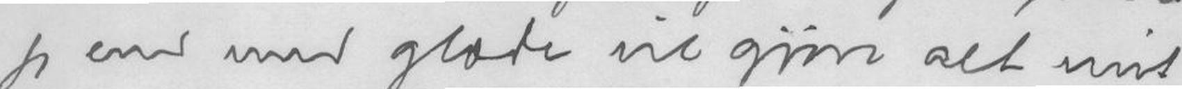jeg end med glæde vil gjøre alt mit
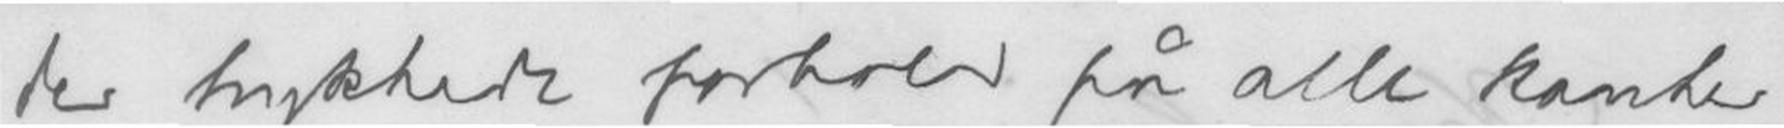der trykkede forhold på alle kanter
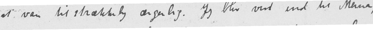at være tilstrækkelig ærgerlig. Jeg blev vist ind til Meiner,
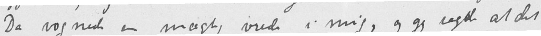Da vognede en mægtig vrede i mig, og jeg sagde at det
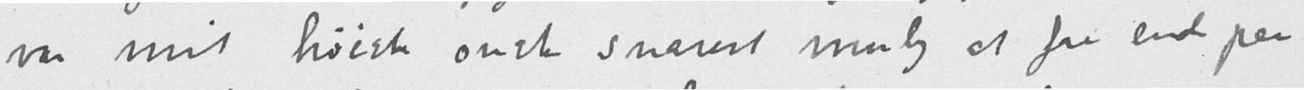var mit høieste ønske snarest mulig at faa ende paa
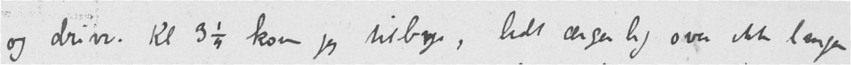og drive. Kl 3 ¼ kom jeg tilbage, lidt ærgerlig over ikke længer
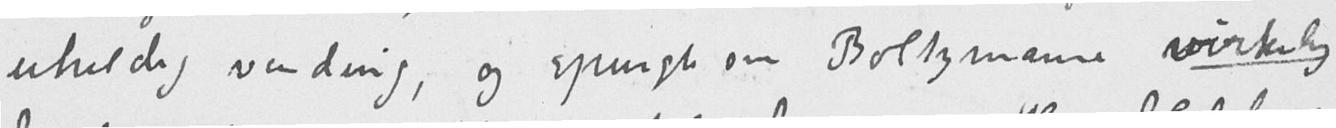uheldig vending, og spurgte om Boltzmann virkelig
